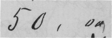50,00
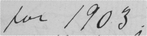for 1903.
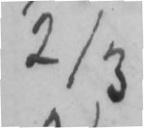2/3
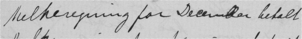Melkeregning for December betalt
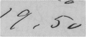19.50
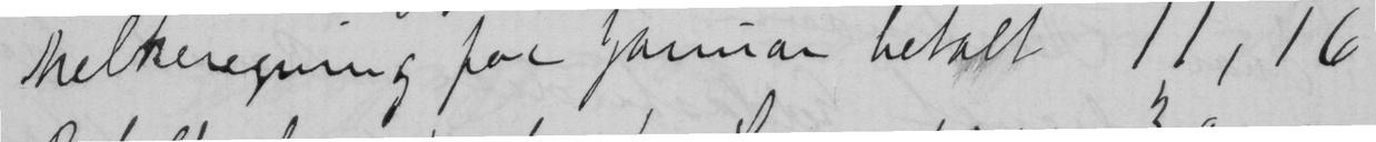Melkeregning for Januar betalt 11,16
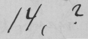14, ?
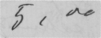5,00
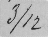3/12
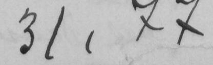31, 77
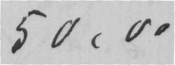50, 00
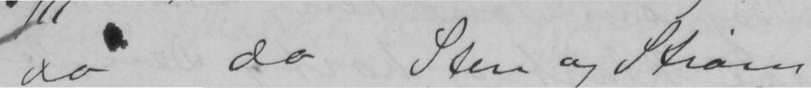xo do Sten og Strøm
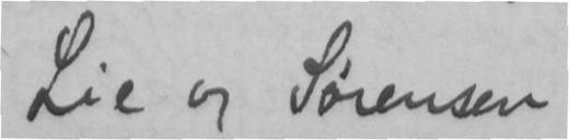Lie og Sørensen
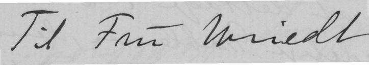Til Fru Wriedt
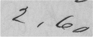2.60
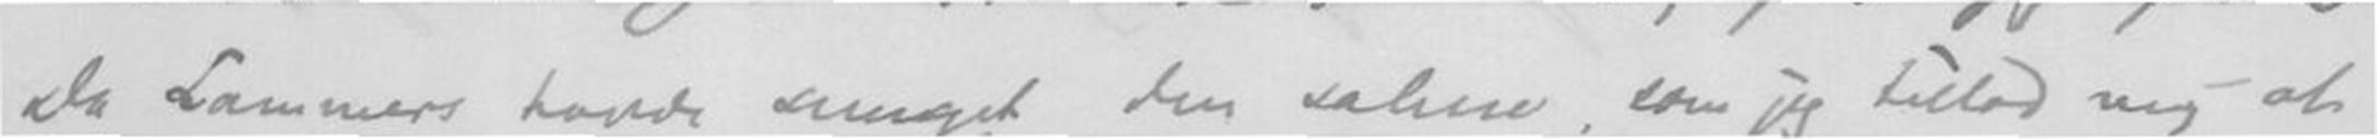Da Lammers havde sunget den salme som jeg tillod mig at
